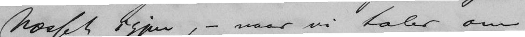Næsset igjen, - naar vi taler om
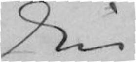Din
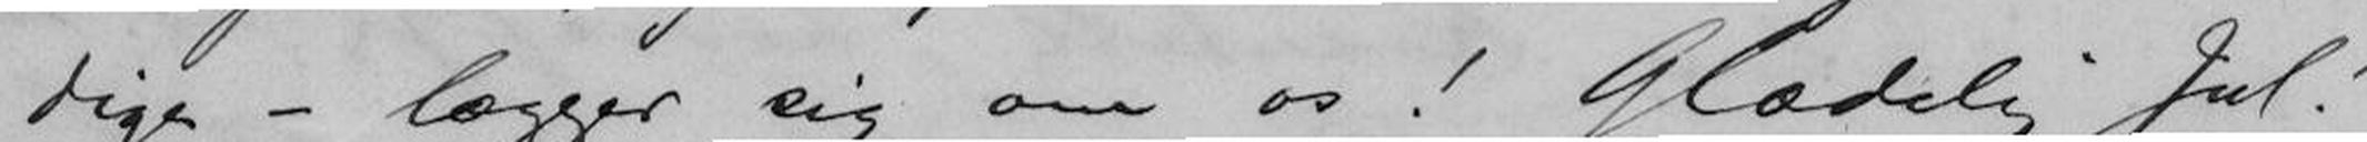dige - lægger sig om os! Glædelig Jul!
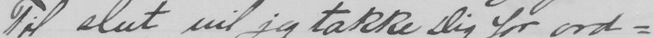Til slut vil jeg takke Dig for ord-
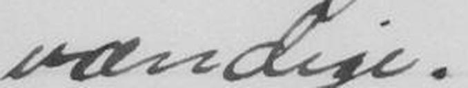vændige.
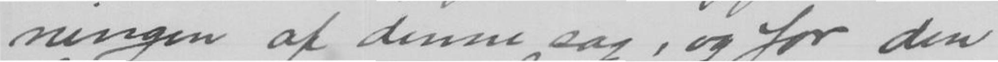ningen af denne sag, og for den
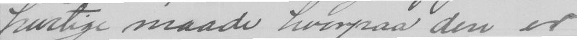hurtige maade hvorpaa den er
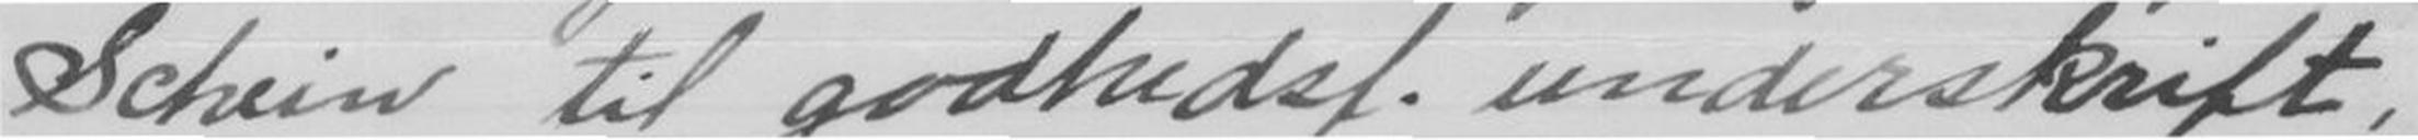Schein til godhedsf. underskrift,
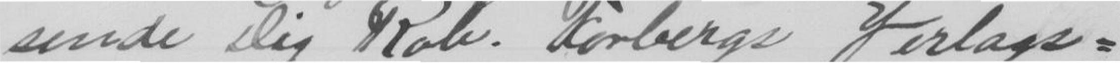sende Dig Rob. Forbergs Verlags-
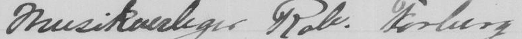Musikverleger Rob. Forberg
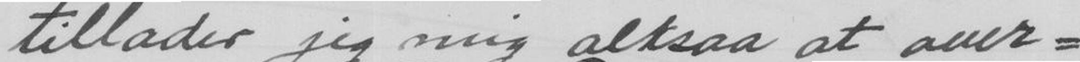tillader jeg mig altsaa at over-
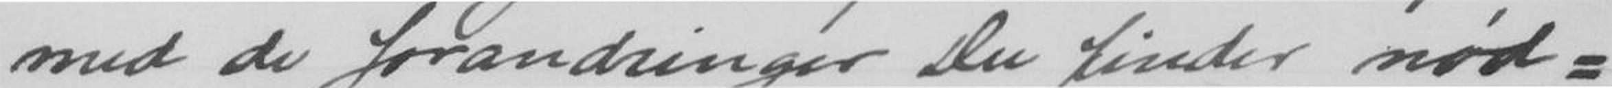med de forandringer Du finder nød-
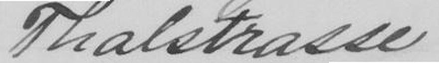Thalstrasse
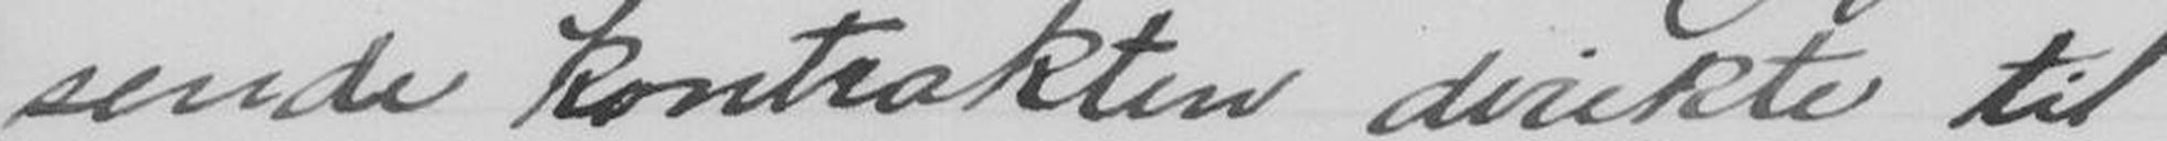sende kontrakten direkte til
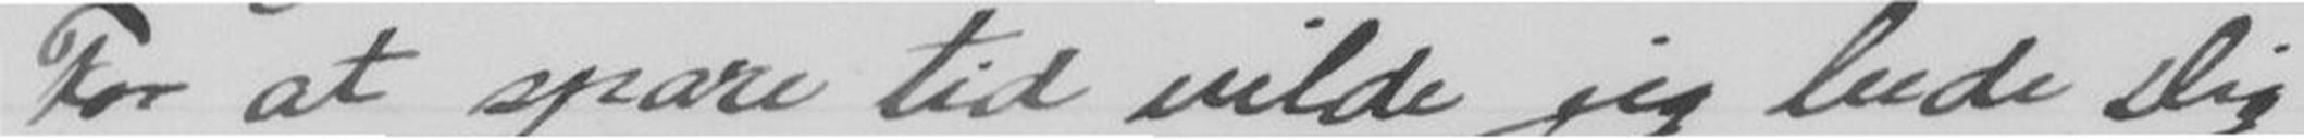For at spare tid vilde jeg bede Dig
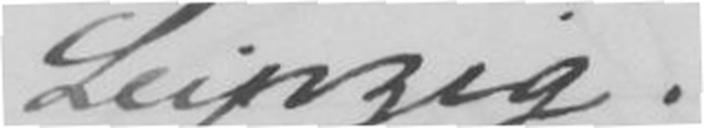Leipzig.
